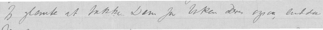Jeg glemte at takke Dem for boken Deres ogsaa, endda
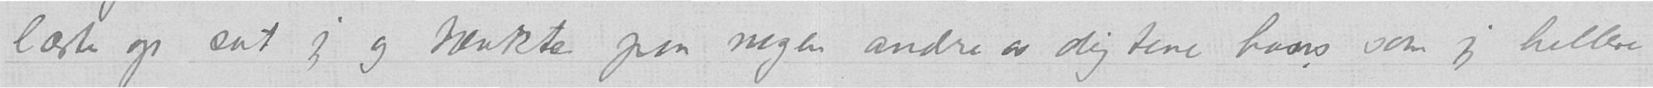læste op sat jeg og tænkte paa nogen andre av digtene hans som jeg hellere
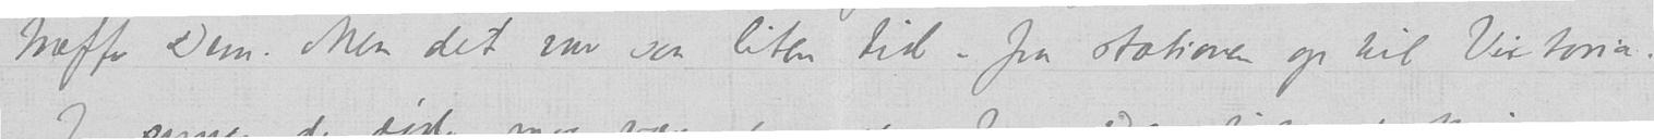træffe Dem. Men det var saa liten tid – fra stationen op til Victoria.
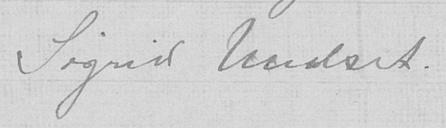Sigrid Undset.
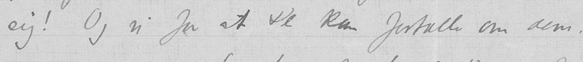sig. Og vi for at De kan fortælle om dem.
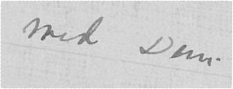med Dem.
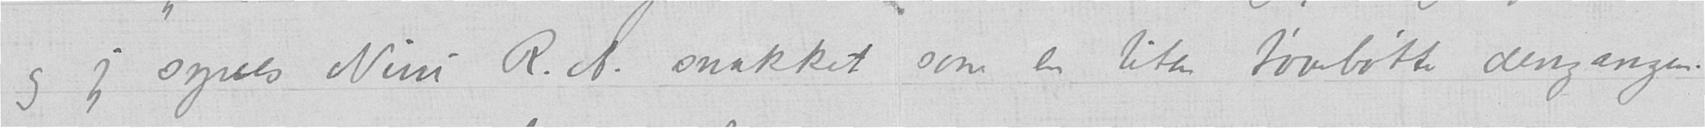og jeg synes Nini R.A. snakket som en liten tøvbøtte dengangen.
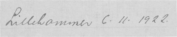Lillehammer 6.11. 1922.
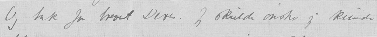Og tak for brevet Deres. Jeg skulde ønske jeg kunde
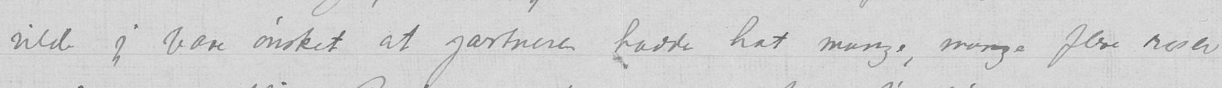vilde jeg bare ønsket at gartneren hadde hat mange, mange flere roser
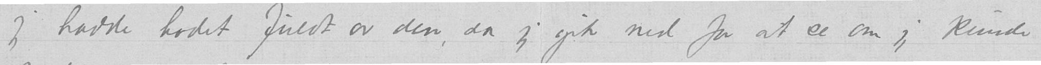jeg hadde hodet fuldt av den, da jeg gik ned for at se om jeg kunde
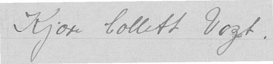Kjære Collett Vogt,
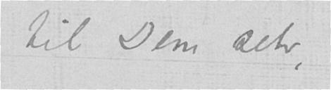til Dem selv,
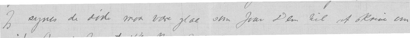Jeg synes de døde maa være glae som faar Dem til at skrive om
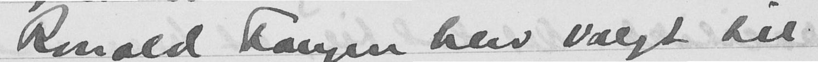Ronald Fangen blev valgt til
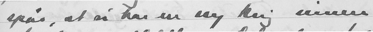spår, at vi har en ny krig innen
